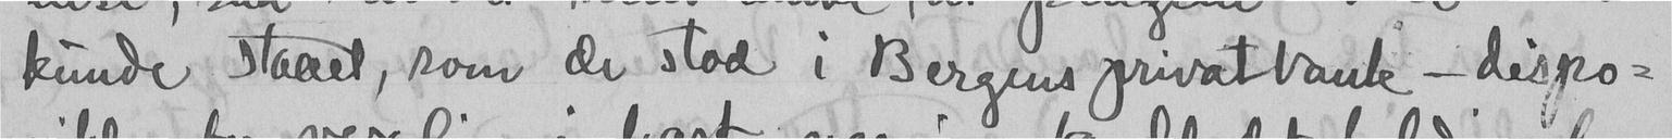kunde staaet, som de stod i Bergens privatbank - dispo-
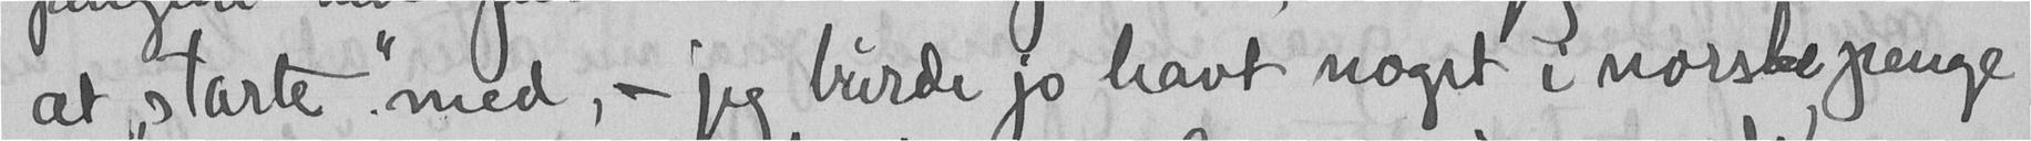at "starte" med, - jeg burde jo havt noget i norske penge
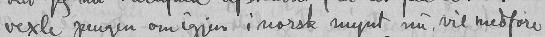vexle pengen om igjen i norsk mynt nu, vil medføre
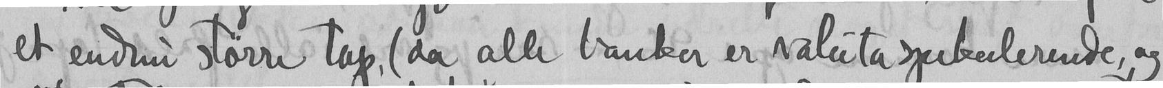et endnu større tap, (da alle banker er valutaspekulerende, og
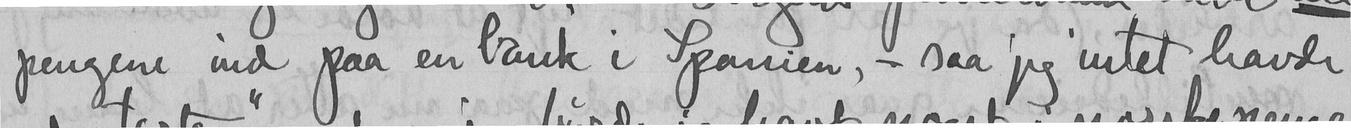pengene ind paa en bank i Spanien, - saa jeg intet havde
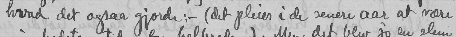hvad det ogsaa gjorde; - (det pleier i de senere aar at være
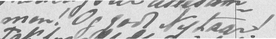men. Og godt Nytaar!
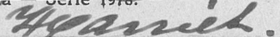Harriet.
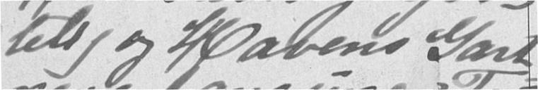telig og Havens Gart-
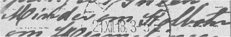Minder om Kolbotn
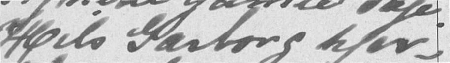Hils Garborg hjer-
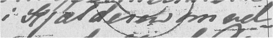i Kjælderen om vel-
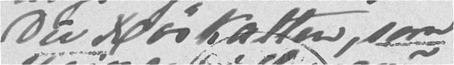Du Røiskatten, som
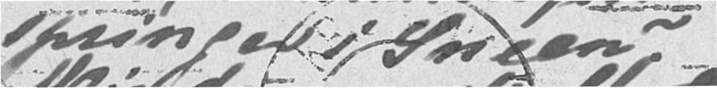springer i Sneen?
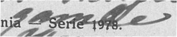gamle
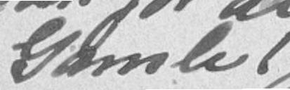Gamle!
